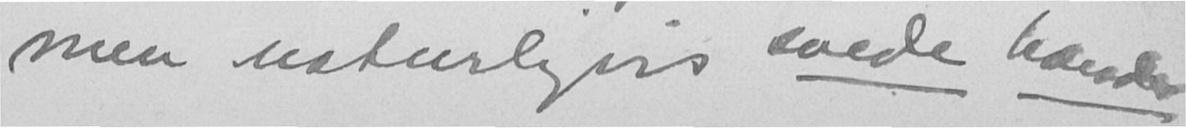men naturligvis svede hænder
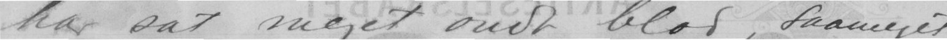har sat meget ondt blod saameget
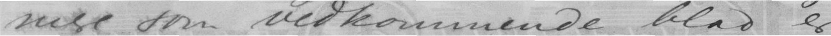mere som vedkommende blad er
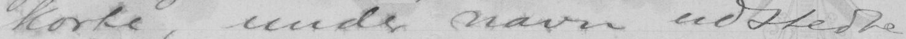korte, under navn udstedte
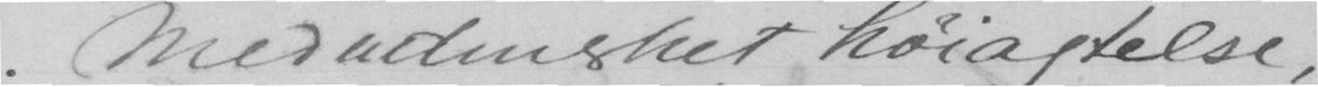Med udmerket høiagtelse,
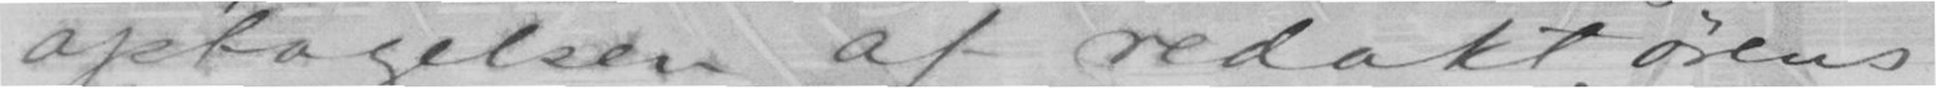optagelsen af redaktørens
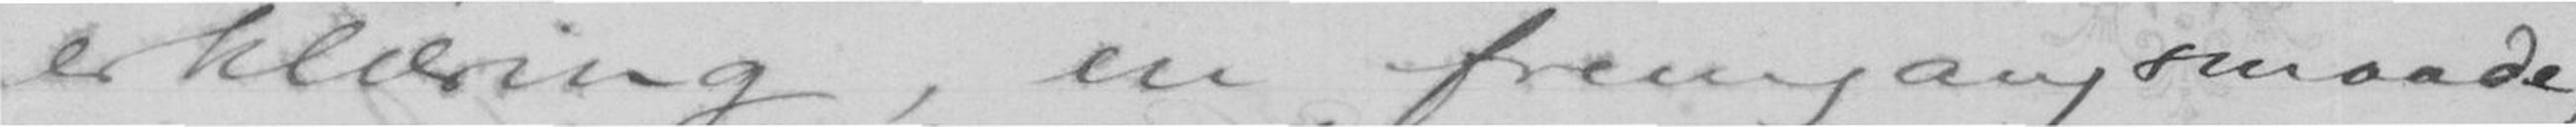erklæring, en fremgangsmaade,
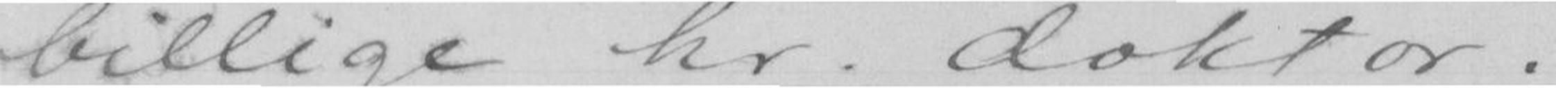billige hr. doktor.
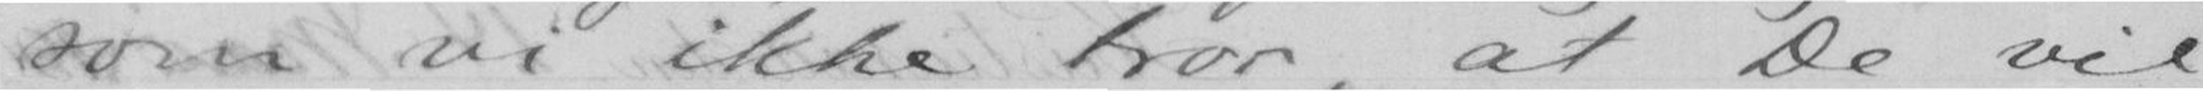som vi ikke tror, at De vil
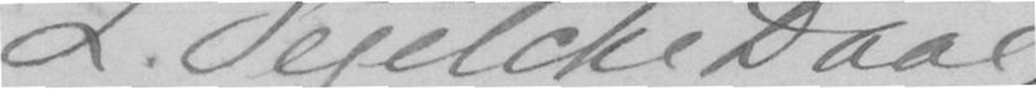L. Segelcke Daae
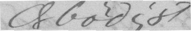Ærbødigst
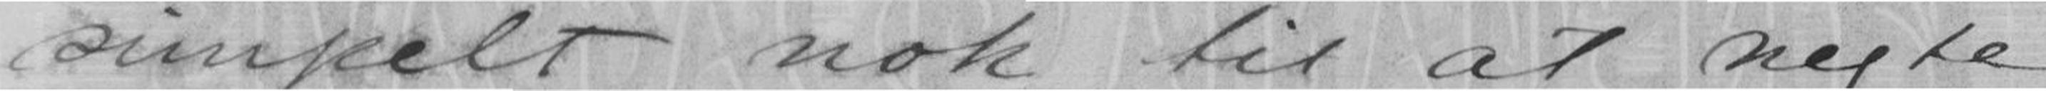simpelt nok til at negte
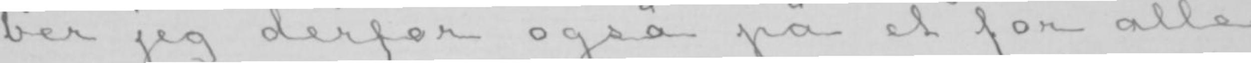ber jeg derfor også på et for alle
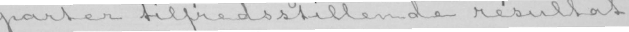parter tilfredsstillende resultat
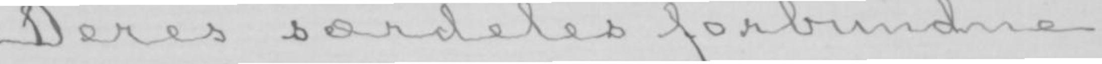Deres særdeles forbundne
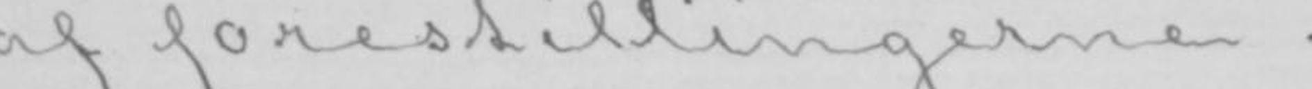af forestillingerne.
